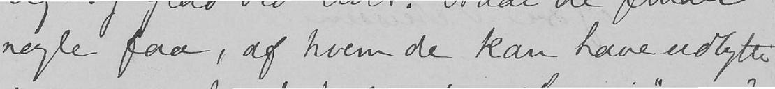nogle faa, af hvem de kan have udbytte
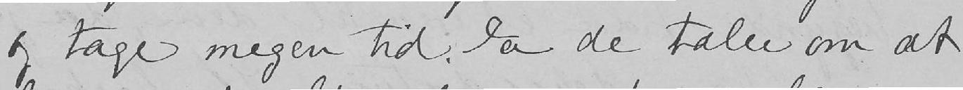og tage megen tid. Ja de taler om at
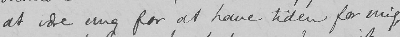at være ung for at have tiden for mig,
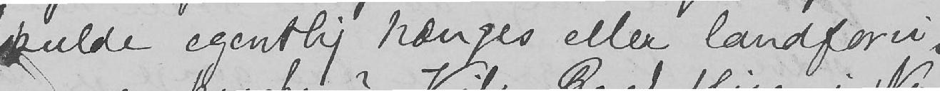skulde egentlig hænges eller landforvi
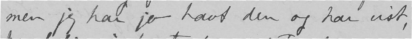men jeg har jo havt den og har vist,
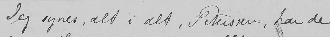Jeg synes, alt i alt, Peterssen, har de
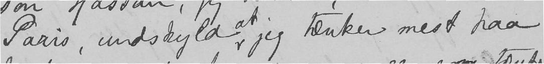Paris, undskyld at jeg tænker mest paa
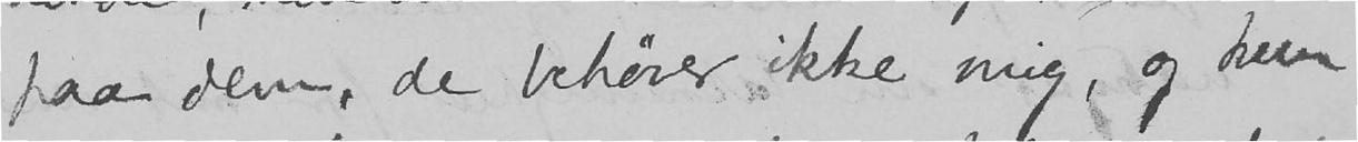paa dem, de behøver ikke mig, og hun
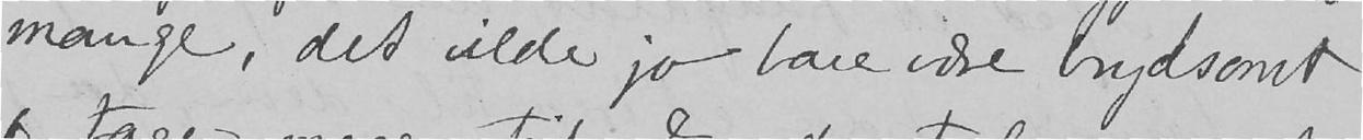mange, det vilde jo bare være brydsomt
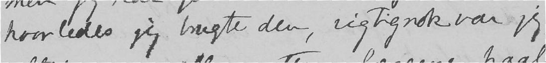hvorledes jeg brugte den, riktignok var jeg
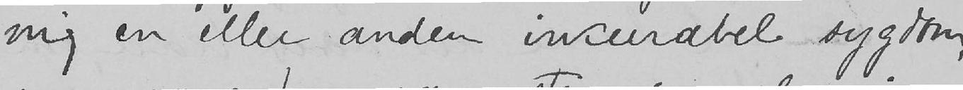mig en eller anden incurabel sygdom,
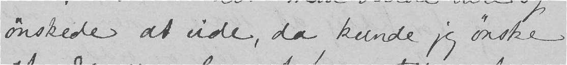ønskede at vide, da kunde jeg ønske
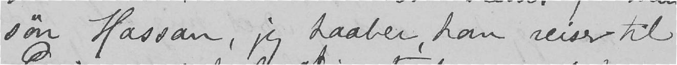søn Hassan, jeg haaber, han reiser til
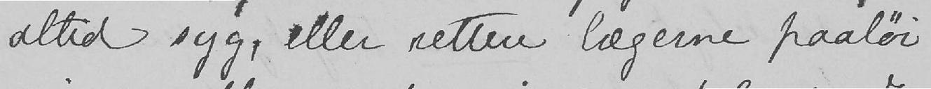altid syg, eller rettere lægerne paaløi
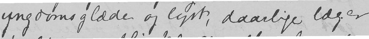ungdomsglæde og lyst, daarlige læger
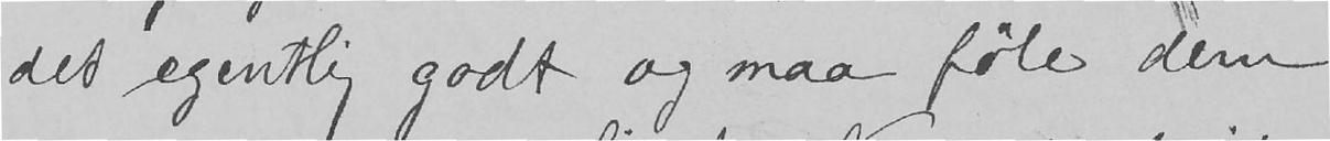det egentlig godt og maa føle dem
